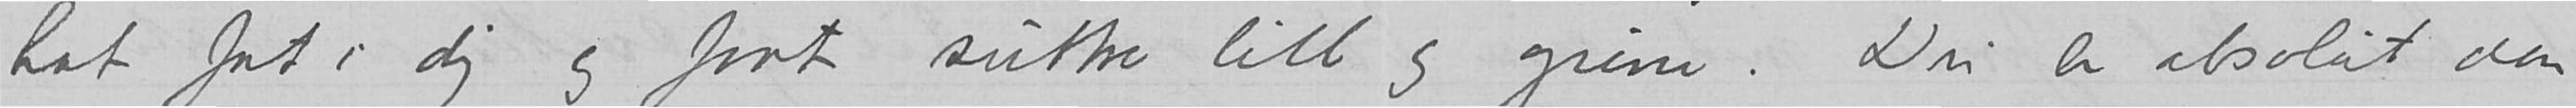hat fat i dig og faat suttre litt og grine. Du er absolut den
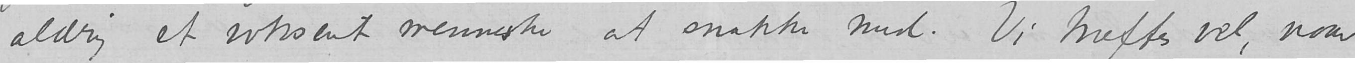aldrig et voksent menneske at snakke med. Vi træffes vel, naar
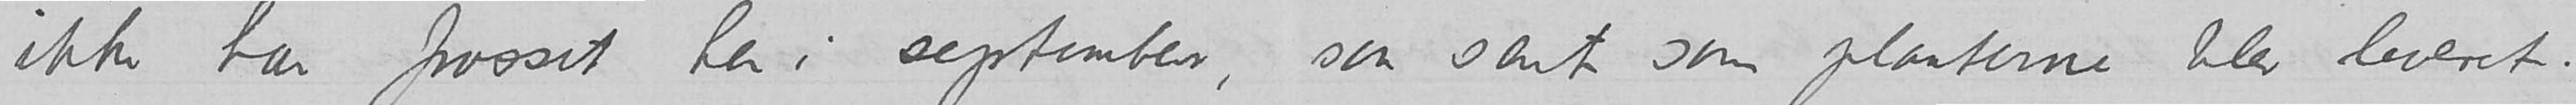ikke har frosset her i september, saa sent som planterne blev leveret.
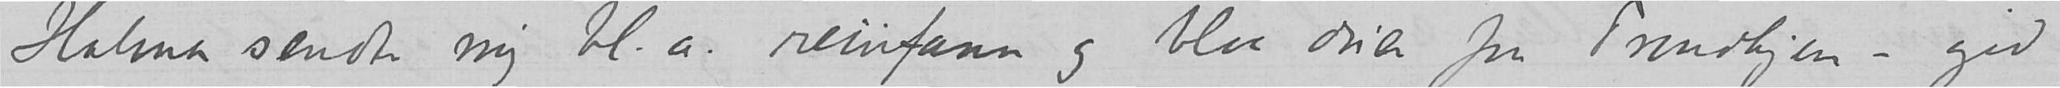Halma sendte mig bl.a. reinfann og blaa druer fra Trondhjem – gid
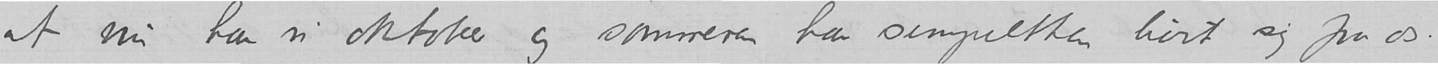at nu har vi oktober og sommeren har simpelthen lurt sig fra os?
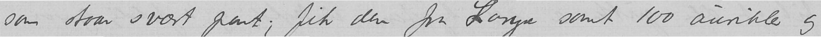som staar svært pent; fik dem fra Lange samt 100 aurikler og
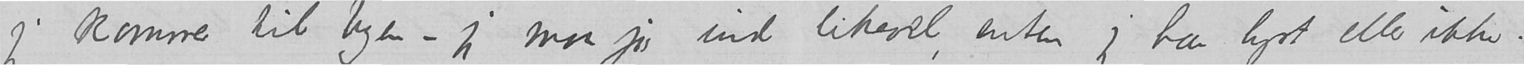jeg kommer til byen – jeg maa ju ind likevel, enten jeg har lyst eller ikke.
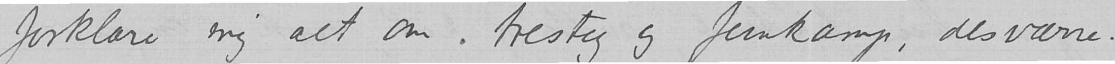forklare mig alt om tresteg og femkamp, desværre.
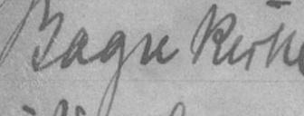Bagn kirke
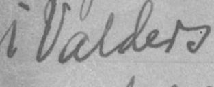i Valdres
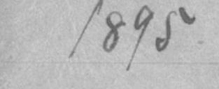1895
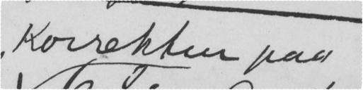Korrektur paa
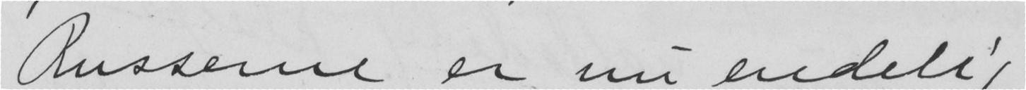Russerne er nu endelig
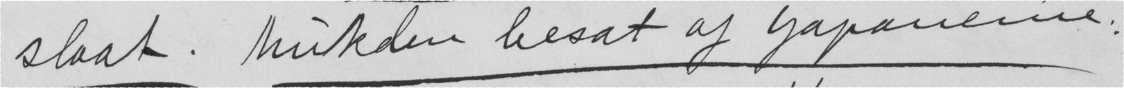slaat. Mukden besat af Japanerne.
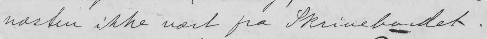næsten ikke vært fra Skrivebordet.
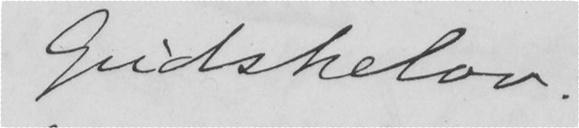Gudskelov.
